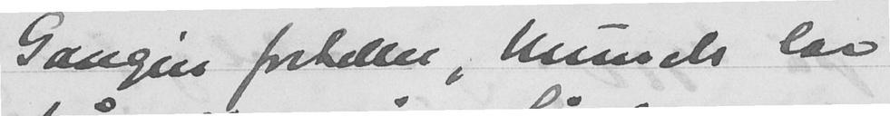Gaugin forteller, Munch lar
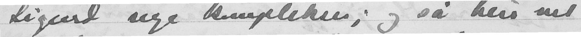Sigurd nye komplekser; og så blir vel
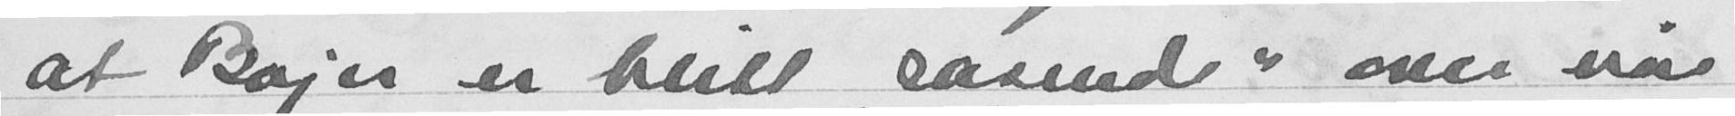at Bojer er blitt "rasende" over vår
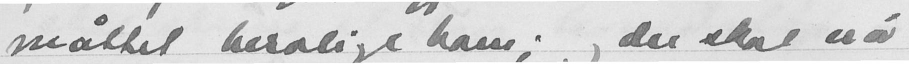måttet berolige ham; og der skal nå
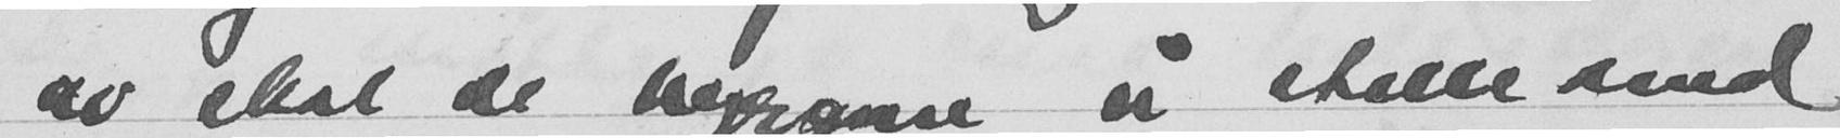av skal de begynne å stille med
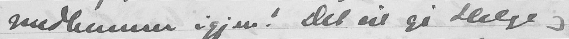medlemmer igjen! Det vil gi Helge og
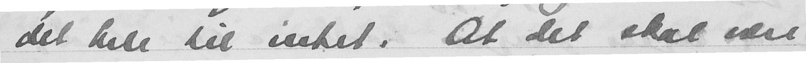det hele til intet. At det skal være
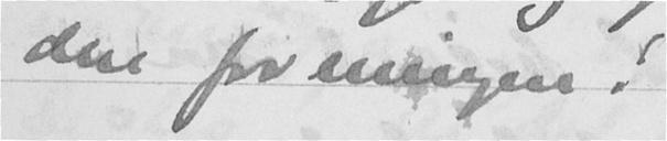den foreningen.
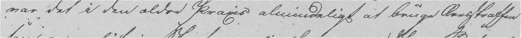var det i den ældre Praxis almindeligt at bruge Ærestraffen
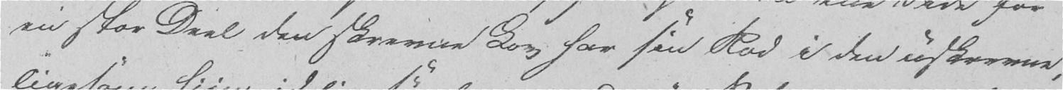en stor Deel den skrevne Lov har sin Rod i den uskrevne,
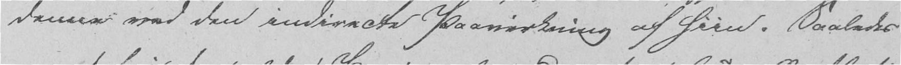denne med den indirecte Paavirkning af hiin. Saaledes
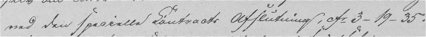ved den specielle Kontracts Afslutning, cfr 3-19-35.
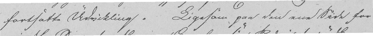fortsatte Udvikling. Ligesom paa den ene Side for
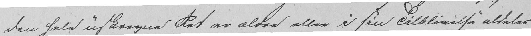den hele uskrevne Ret er ældre eller i sin Tilblivelse aldeles
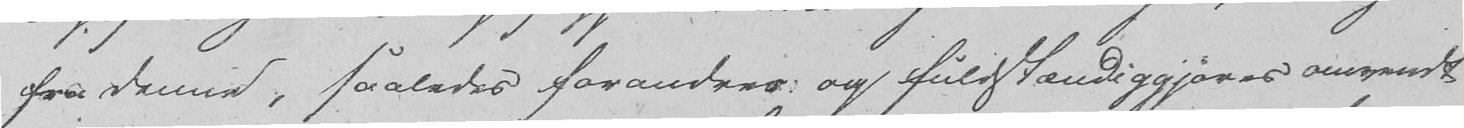fra denne, saaledes forandres og fuldstændiggjøres omvendt
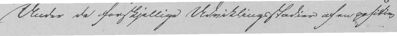Under de forskjellige Udviklingsstadier af en positiv
